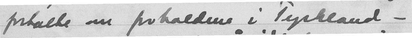fortalte om forholdene i Tyskland -
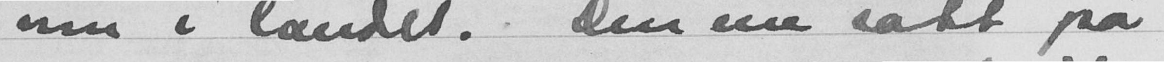inn i landet. Den ene satt på
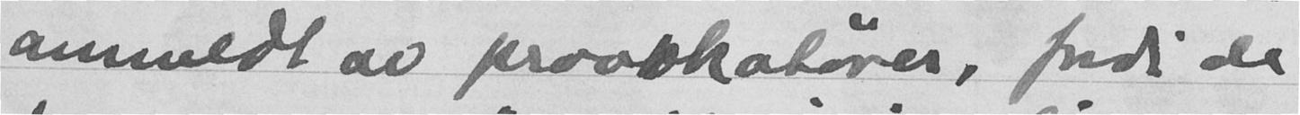anmeldt av provokatører, fordi de
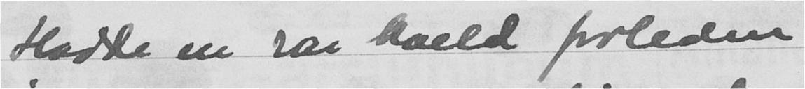Hadde en rar kveld forleden
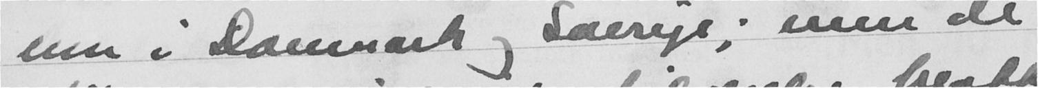enn i Danmmark og Sverige, men de
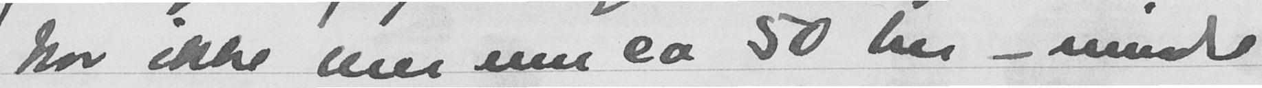har ikke mer enn ca 50 her - mindre
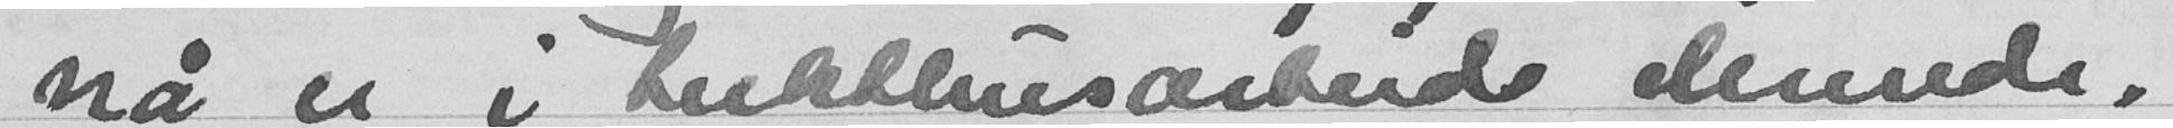nå er i buktlus arbeide dernede,
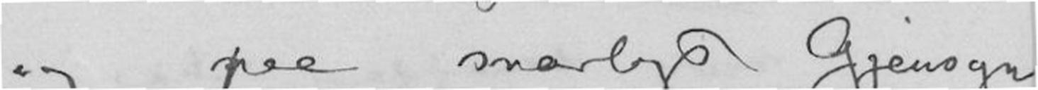og paa snarligt Gjensyn.
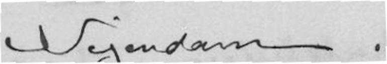Nejendam.
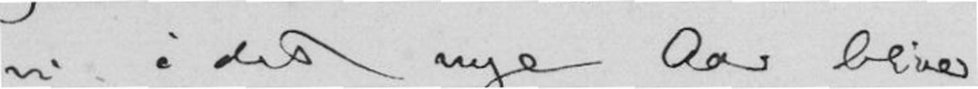vi i det nye Aar bliver
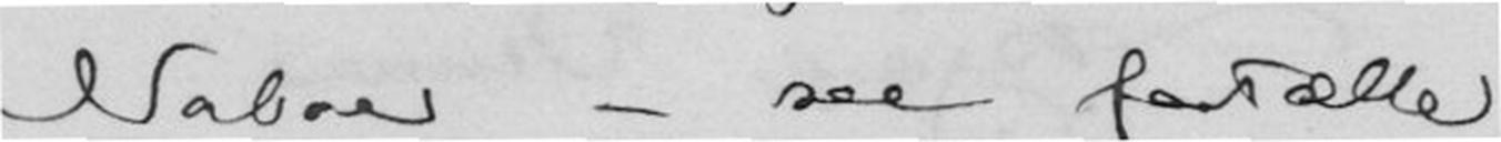Naboer - saa fortælle
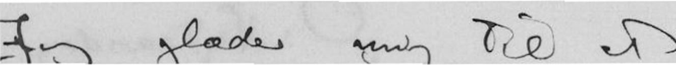Jeg glæder mig til at
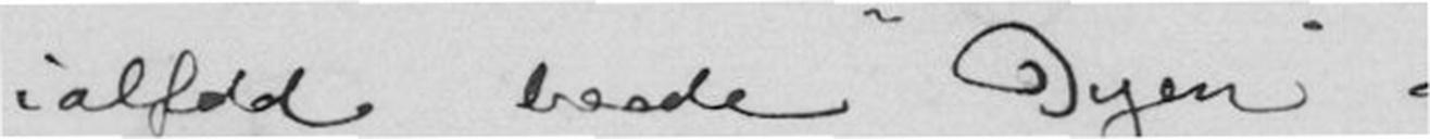ialfald baade "Byen" og
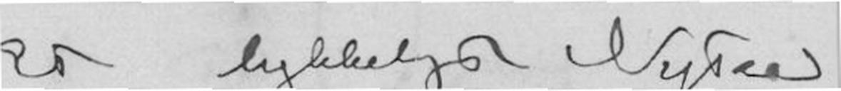Et lykkeligt Nytaar
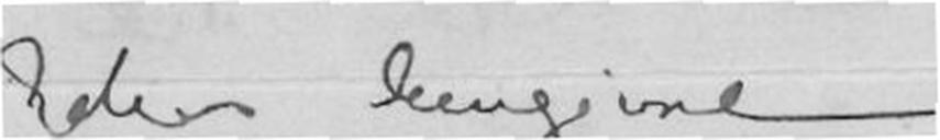Eders hengivne
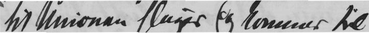til Unionen sluger og kommer til
